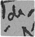de
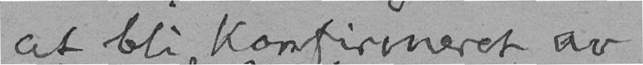at bli Konfirmeret av
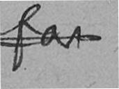for
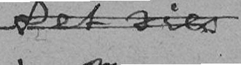Det sies
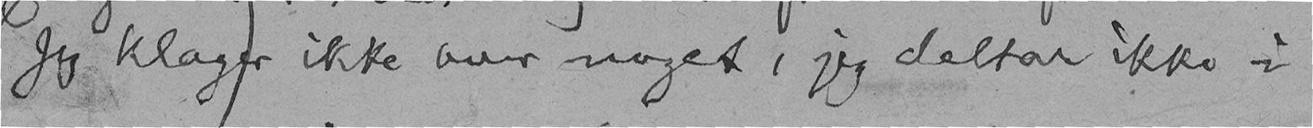Jeg klager ikke over noget, jeg deltar ikke i
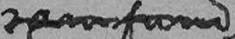sanrsfumd
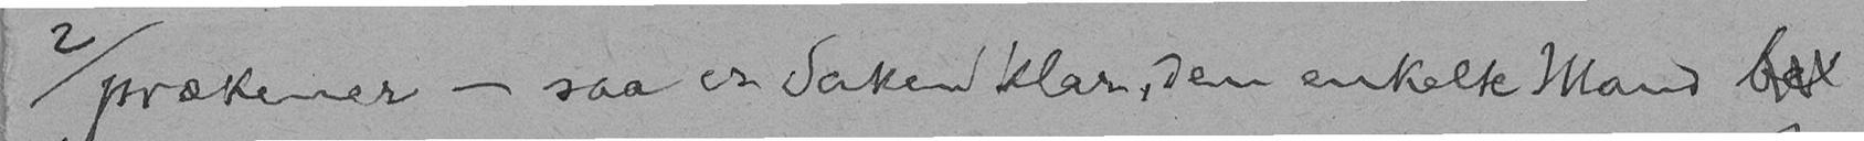prækener - saa er Saken klar, den enkelte Mand be
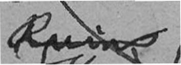Ruin
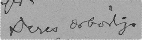Deres ærbødige
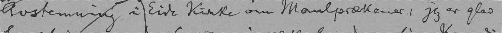Avstemning i Eide Kirke om Maulprækener, jeg er glad
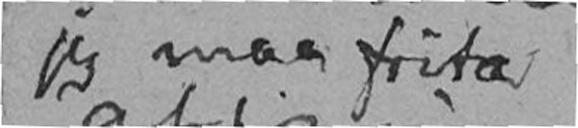jeg maa frita
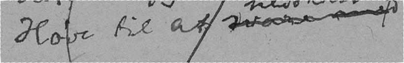Høve til at svare med
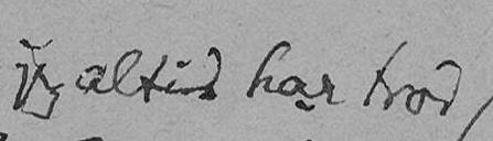jeg altid har trod
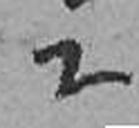i
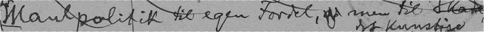Maulpolitik til egen Fordel, og men til Skade
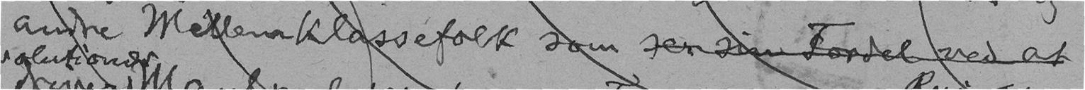andre Mellemklassefolk som ser sin Fordel ved at
